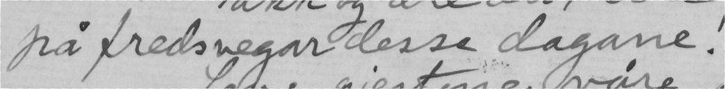på fredsvegar desse dagane!
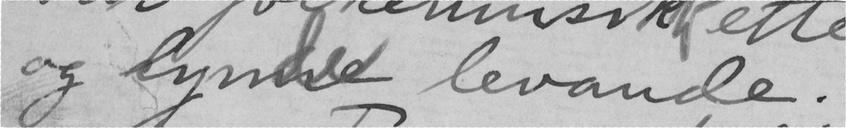og lynde levande.
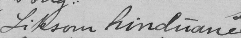Liksom hinduane
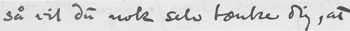så vil du nok selv tænke dig, at
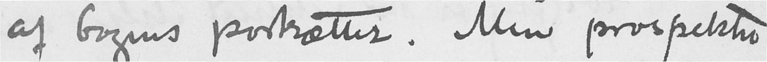af bogens portrætter. Men prospektet
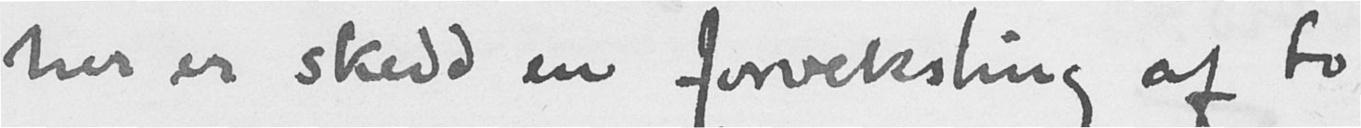her er skedd en forveksling af to
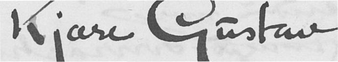Kjære Gustav
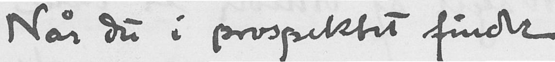Når du i prospektet finder
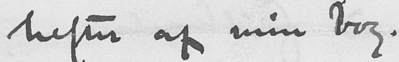hefter af min bog.
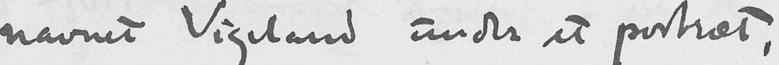navnet Vigeland under et portræt,
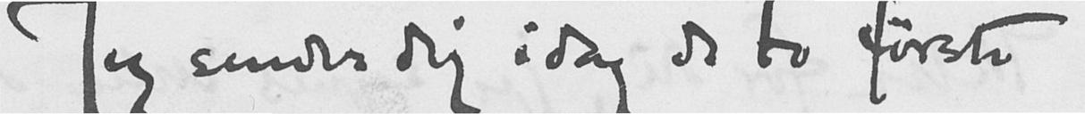Jeg sender dig idag de to første
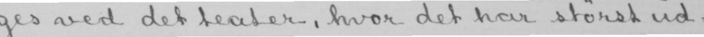ges ved det teater, hvor det har størst ud-
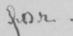for.
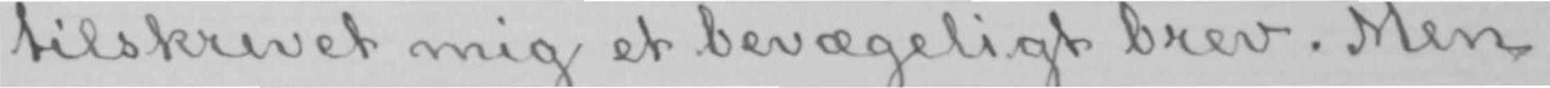tilskrevet mig et bevægeligt brev. Men
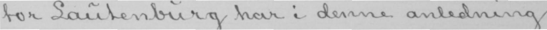tor Lautenburg har i denne anledning
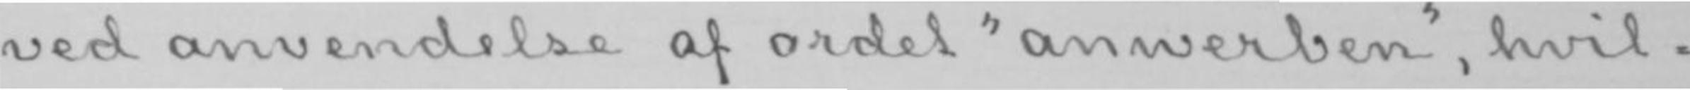ved anvendelse af ordet «anwerben», hvil-
